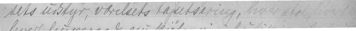sets udstyr, værelsets tapetsering, hver stol, hvert
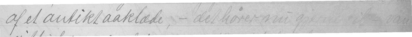af et antikt aaklæde, - det hører nu gjerne til - van-
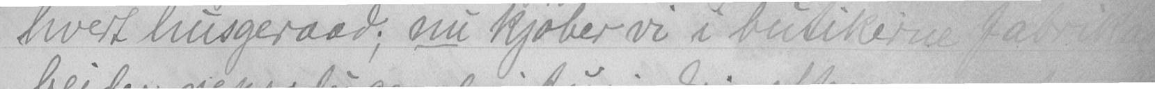hvert husgeraad; nu kjøber vi i butikerne fabrikkar-
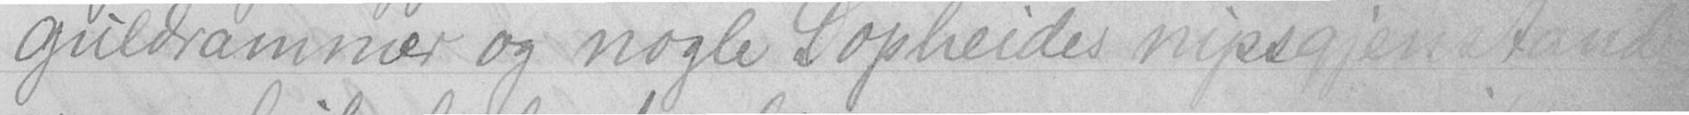guldrammer og nogle Dopheides nipsgjenstander,
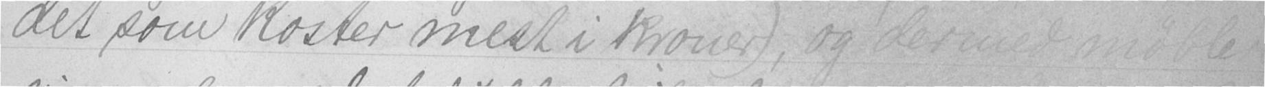det som koster mest i kroner), og dermed møblerer
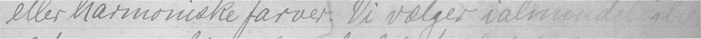eller harmoniske farver. Vi vælger ialmindelighed
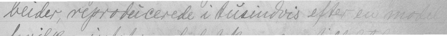beider, reproducerede i tusindvis efter en model, thi
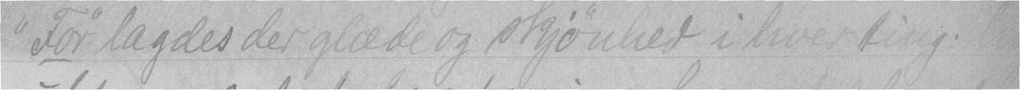"Før lagdes der glæde og skjønhed i hver ting. hu-
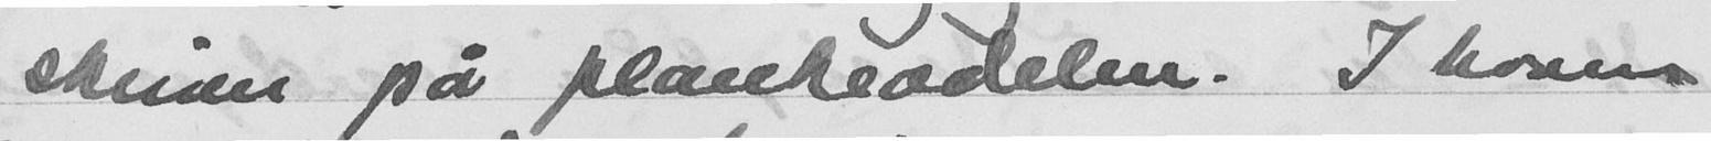skriver på plankeadelen. I haven
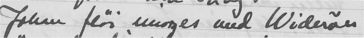Johan fløi imorges med Widerøes
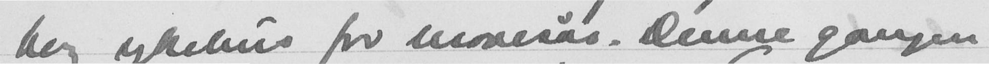berg sykehus for mavesår. Denne gangen
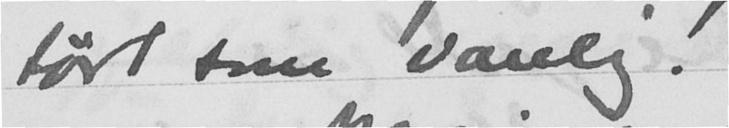tørt som vanlig.
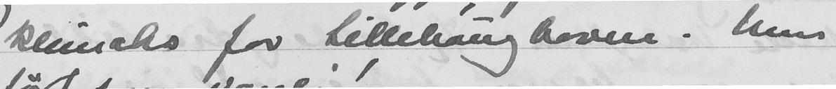kluncks for Lillehaug haven. Men
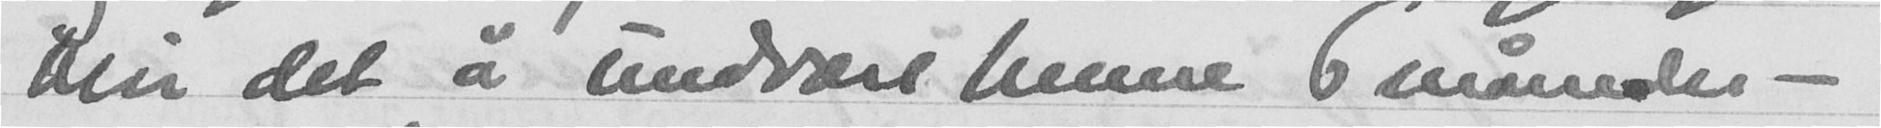blir det å undvære henne 6 måneder -
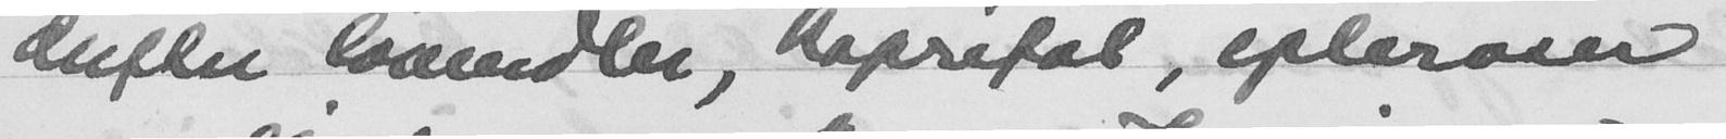dufter lavendler, kaprifol, eplerosen
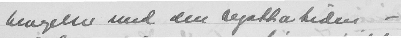bevegelser med den regattatiden -
